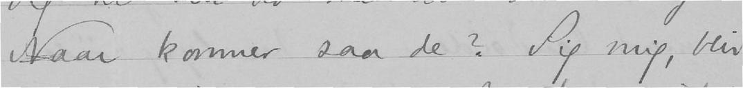Naar kommer saa de? Sig mig, blir
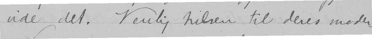vide det. Venlig hilsen til deres moder.
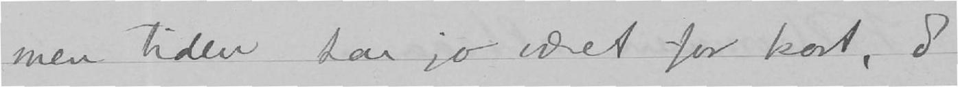men tiden har jo været for kort, 8
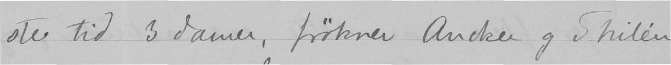ste tid 3 damer, frøkner Ancker og Thilén,
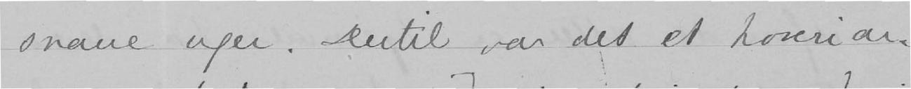snaue uger. Dertil var det et hoveriar-
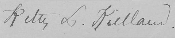Kitty L. Kielland.
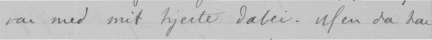var med mit hjerte dabei. Men da har
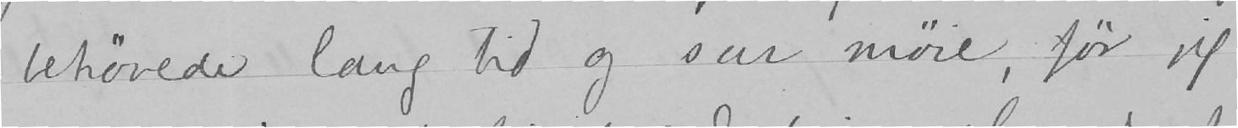behøvede lang tid og sur møie, før jeg
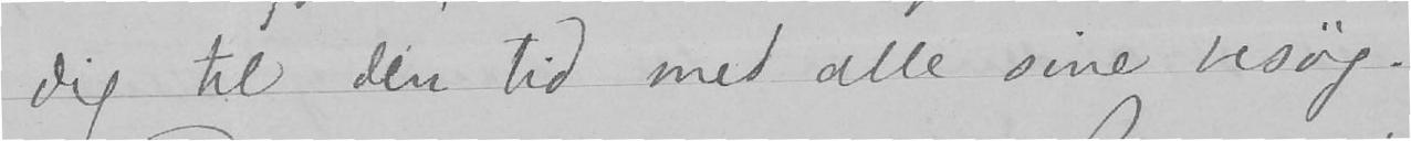dig til den tid med alle sine besøg.
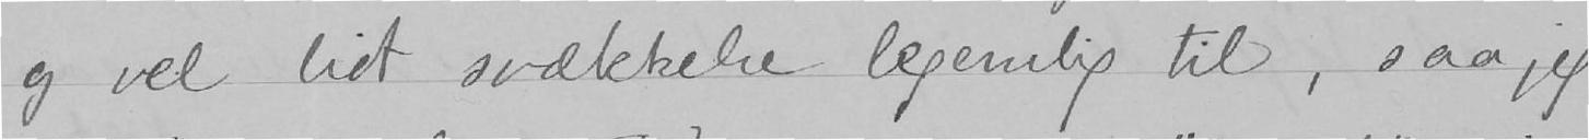og vel lidt svækkelse legemlig til, saa jeg
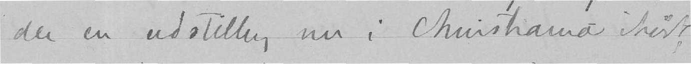der en udstilling, nu i Christiania ihøst
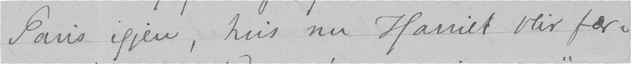Paris igjen, hvis nu Harriet blir fær-
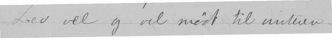Lev vel og vel mødt til vinteren.
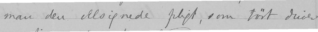man den velsignede pligt, som tørt driver
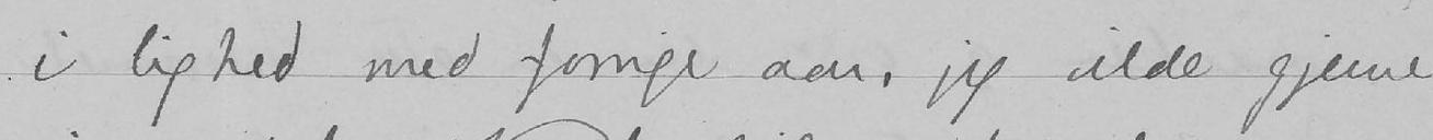i lighed med forrige aar, jeg vilde gjerne
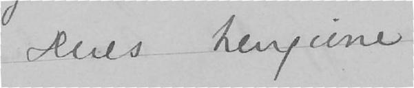Deres hengivne
